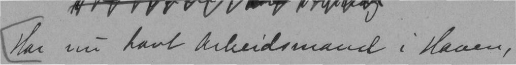Har nu havt arbeidsmand i Haven,
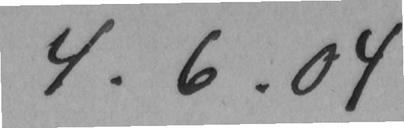4.6.04
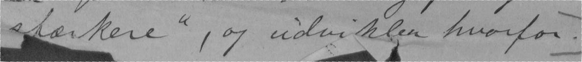stærkere", og udvikler hvorfor.
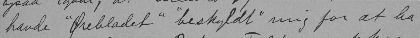havde "Ørebladet" "beskyldt" mig for at ha
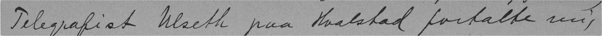Telegrafist Ulseth paa Hvalstad fortalte mig
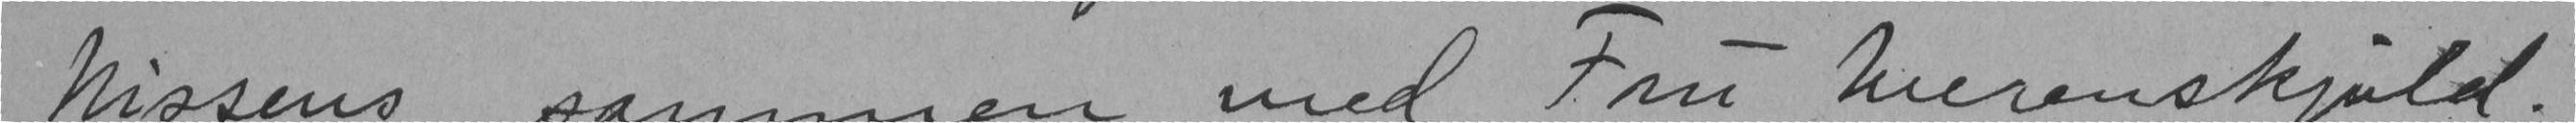Nissens sammen med Fru Werenskjold.
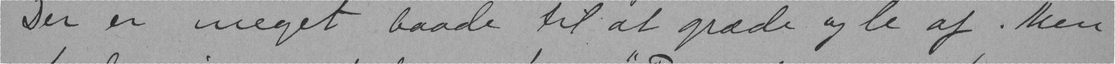Der er meget baade til at græde og le af. Men
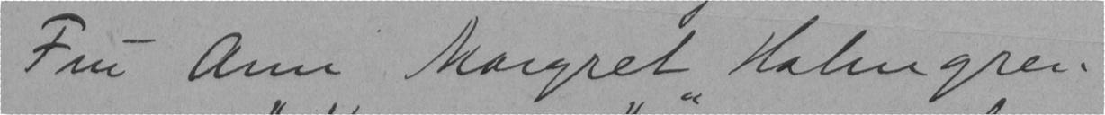Fru Ann Margret Holmgren,
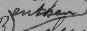entsen
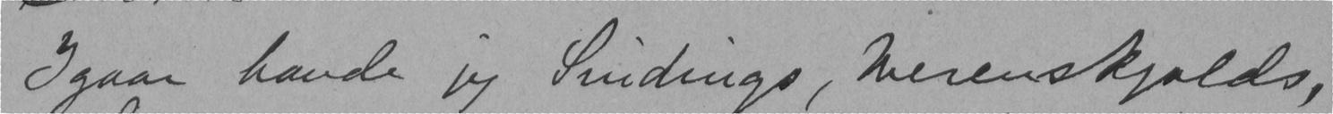Igaar havde jeg Sindings, Werenskjolds,
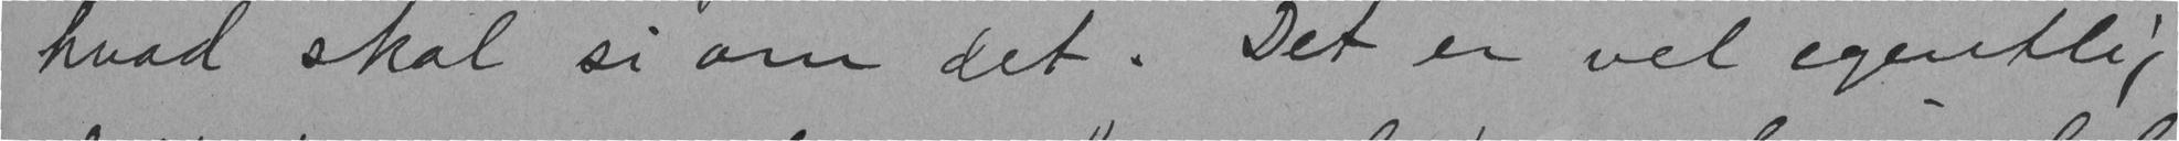hvad skal si om det. Det er vel egentlig
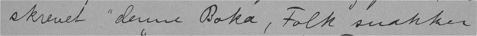skrevet "denne Boka, Folk snakker
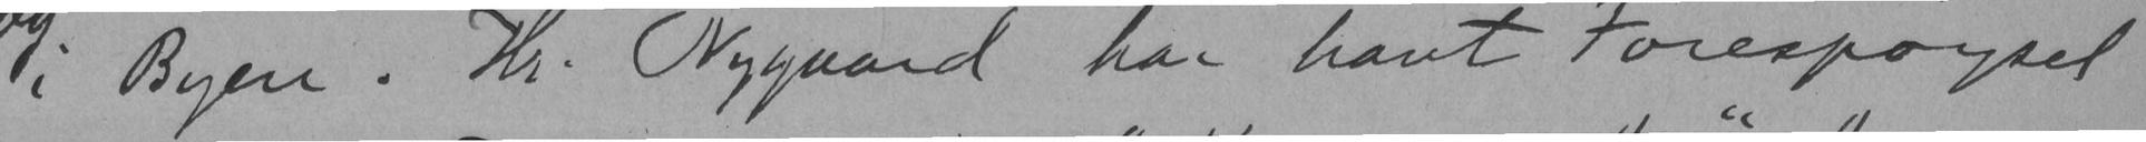i Byen. Hr. Nygaard har havt Forespøgsel
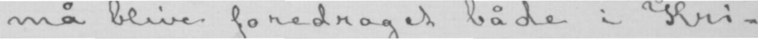må blive foredraget både i Kri-
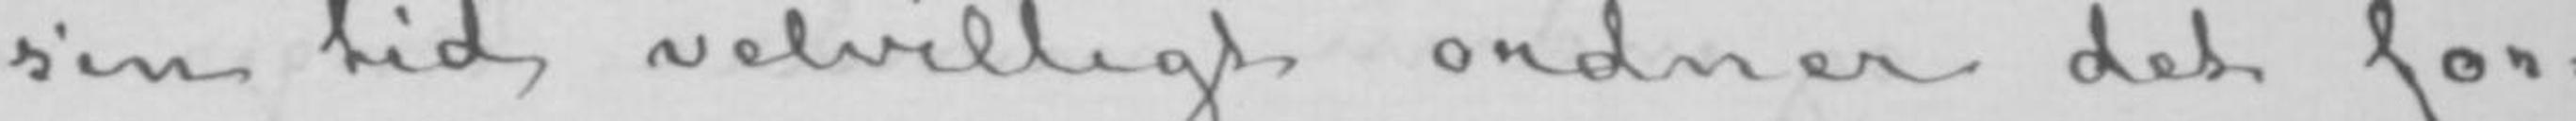sin tid velvilligt ordner det for-
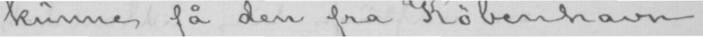kunne få den fra København.
